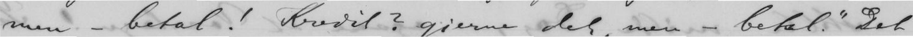men - betal! Kredit? gjerne det, men - betal." Det
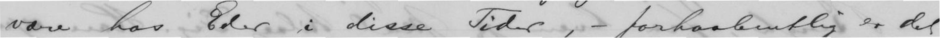være hos Eder i disse Tider, - forhaabentlig er det
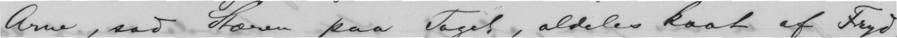Arne, sad Stæren paa Toget, aldeles kaat af Fryd,
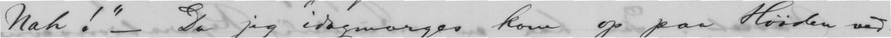"Nah!" - Da jeg idagmorges kom op paa Høiden ved
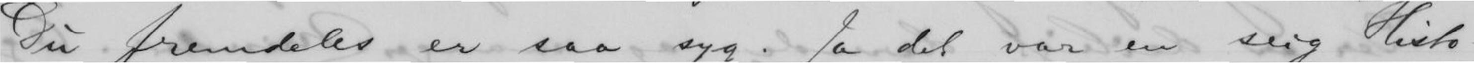Du fremdeles er saa syg. Ja det var en seig Histo
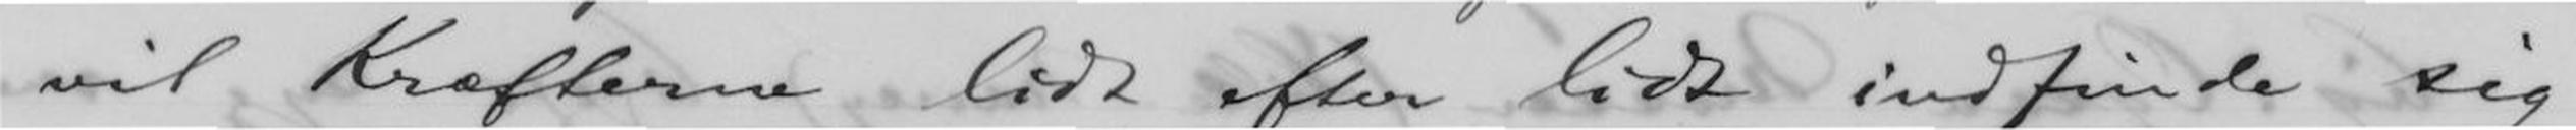gen, saa vil Kræfterne lidt efter lidt indfinde sig.
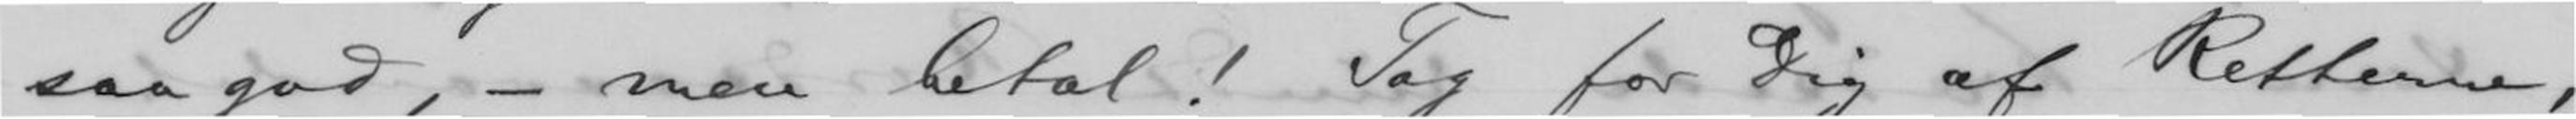saa god, - men betal! Tag for Dig af Retterne,
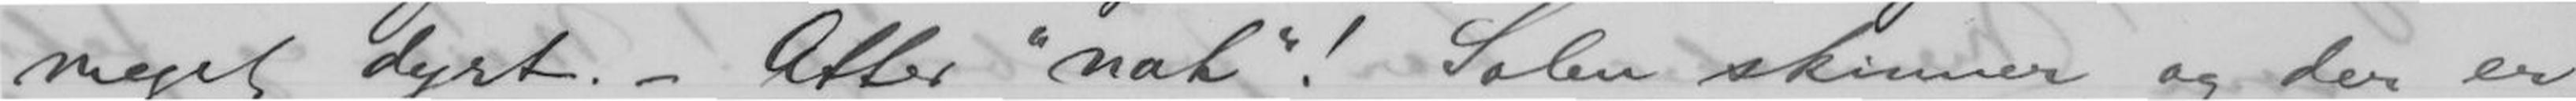meget dyrt. - Atter "nah"! Solen skinner og der er
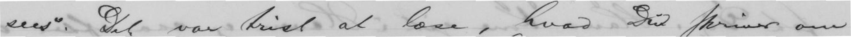sees. Det var trist at læse, hvad Du skriver om
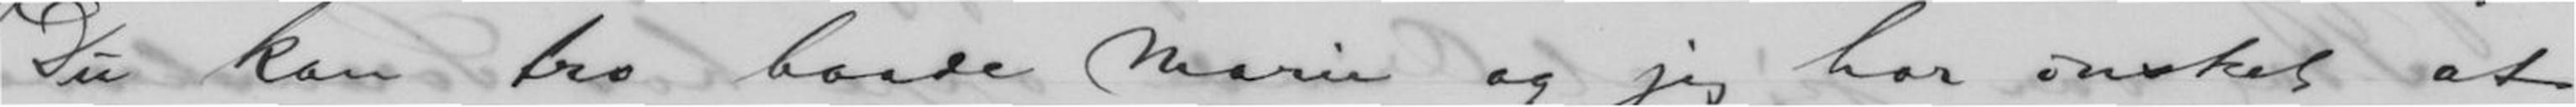Du kan tro baade Marie og jeg har ønsket at
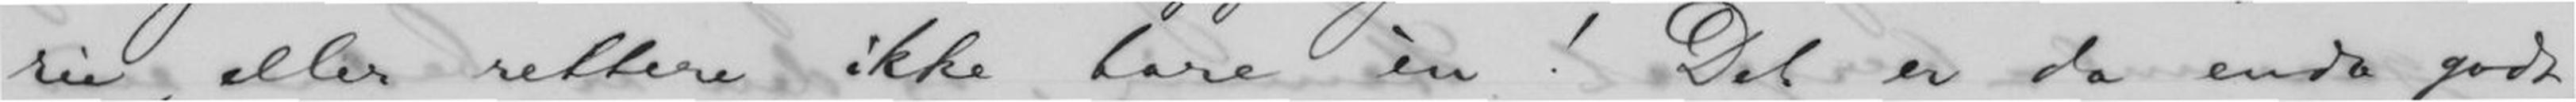rie, eller rettere ikke bare èn! Det er da enda godt,
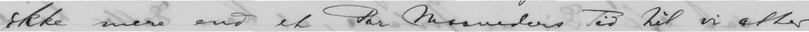ikke mere end et Par Maaneders Tid til vi atter
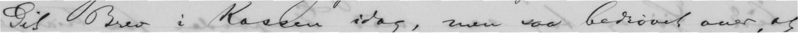Dit Brev i Kassen idag, men saa bedrøvet over, at
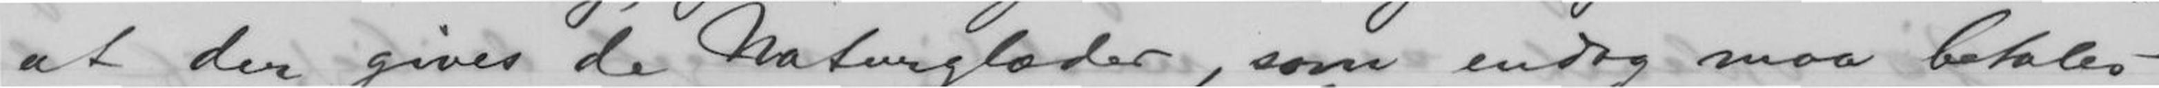at der gives de Naturglæder, som endog maa betales
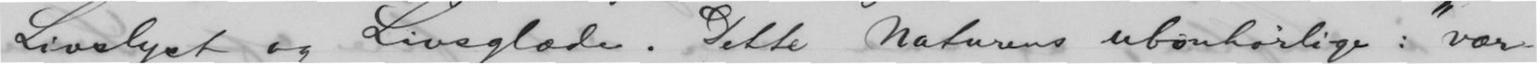Livslyst og Livsglæde. Dette Naturens ubønhørlige: "vær
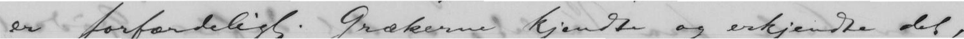er forfærdeligt. Grækerne kjendte og erkjendte det,
